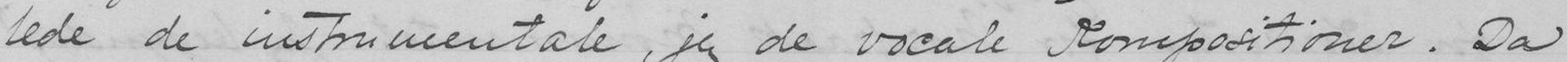lede de instrumentale, jeg de vocale Konpositioner. Da
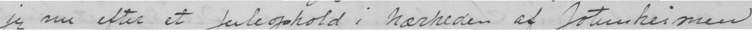jeg nu efter et Juleophold i nærheden af Jotunheimen
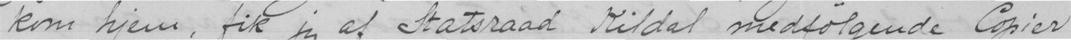kom hjem, fik jeg af Statsraad Kildal medfølgende Copier
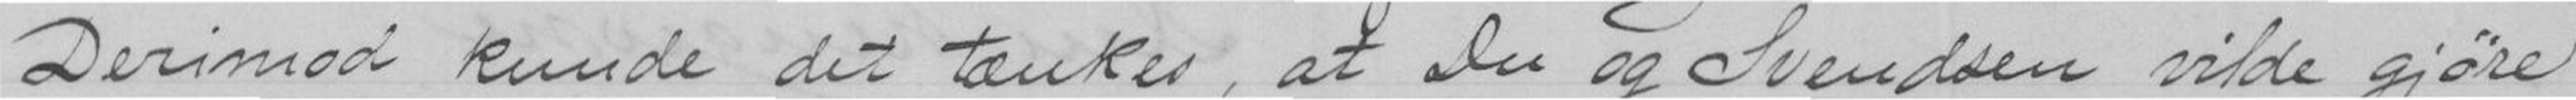Derimod kunde det tænkes, at du og Svendsen vilde gjøre
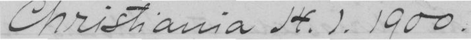Christiania 14.1.1900
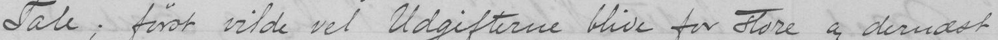Tale; først vilde vel udgifterne blive for Store og dernæst
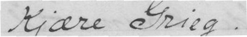Kjære Grieg
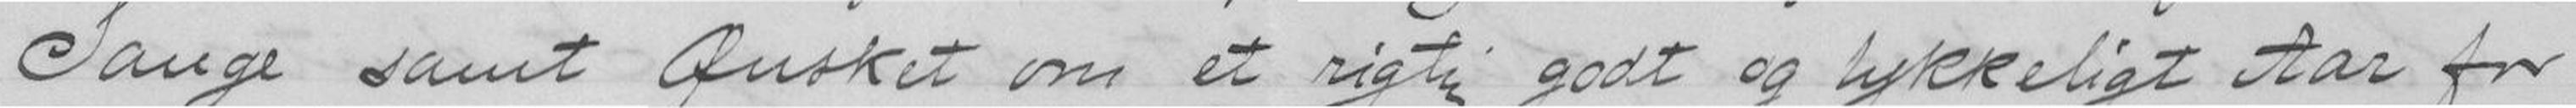Sange samt Ønsket om et rigtig godt og lykkeligt Aar for
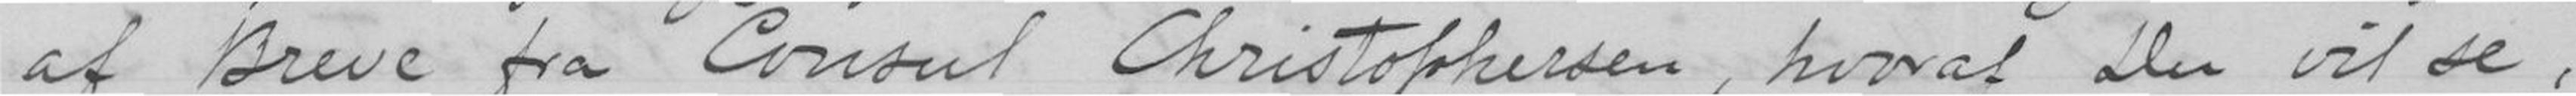af Breve fra Consul Christophersen, hvoraf Den vil se,
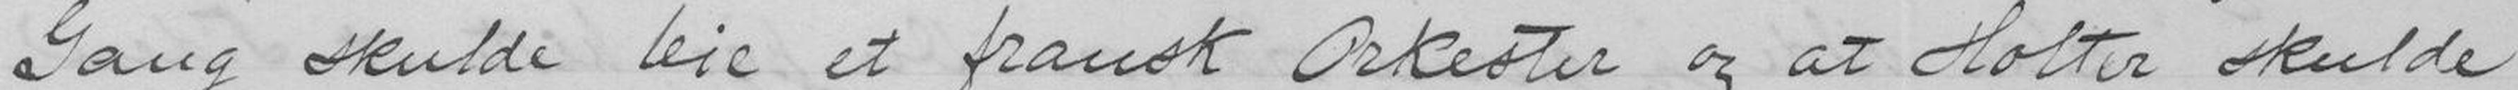Gang skulde leie et fransk Orkester og at Holter skulde
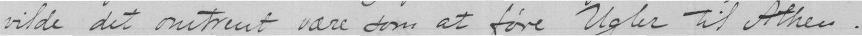vilde det omtrent være som at føre Ugler Til Athen.
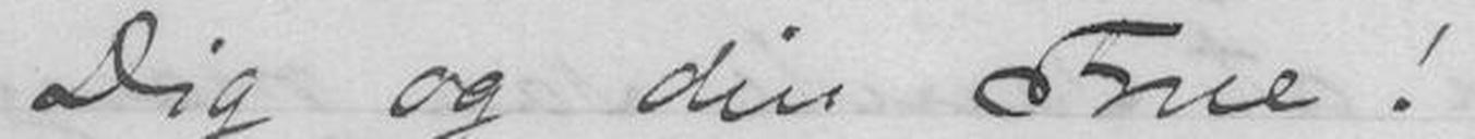Dig og din Frue!
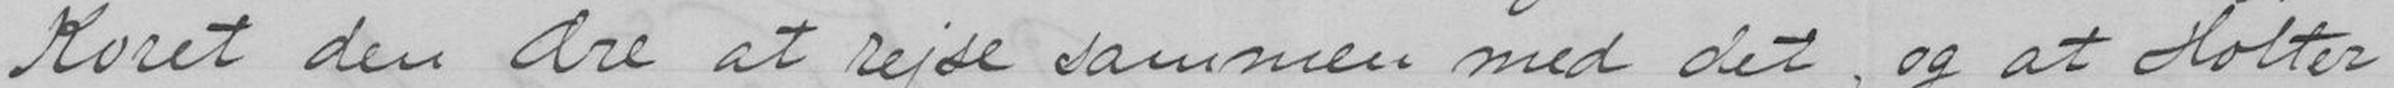Koret den Ære at rejse sammen med det, og at Holter
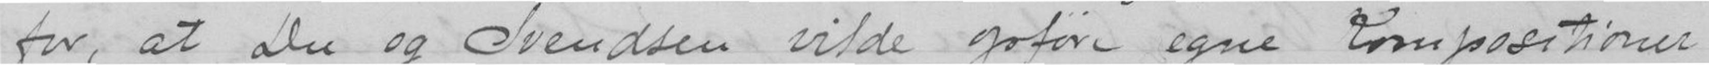for, at Du og Svendsen vilde godføre egne Konpositioner
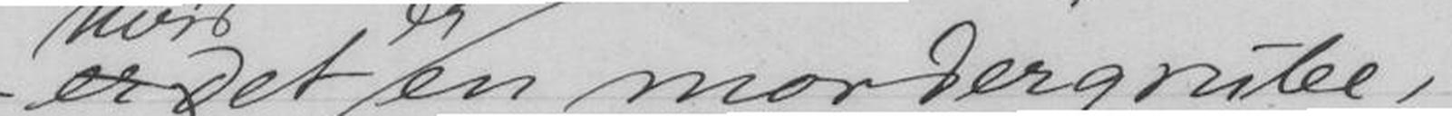er hvis det er en mordergrube,
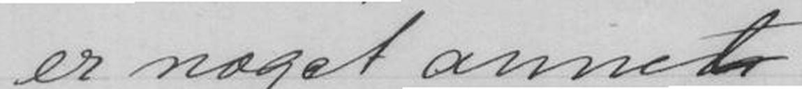er noget annet
